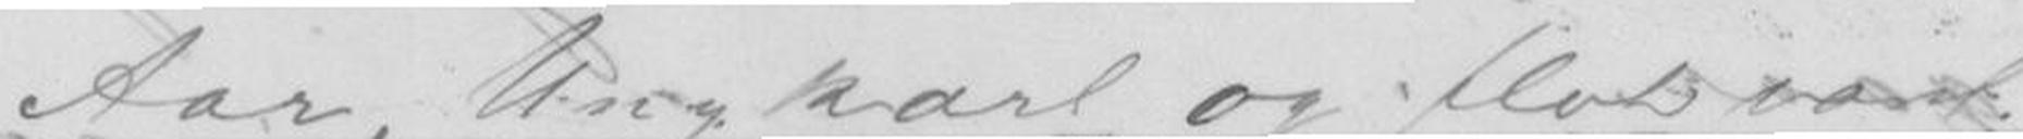40 Aar, Ungkarl og flot vant;
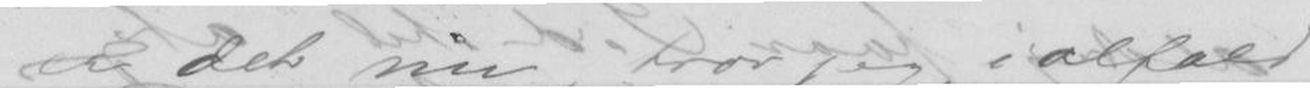er det nu, tror jeg, ialfald
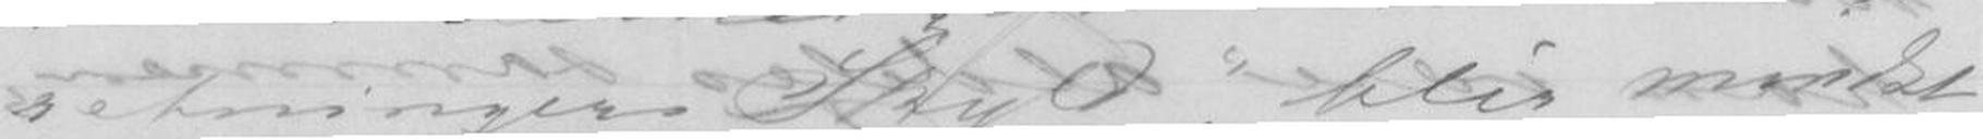retningers Skyld, blir mindst
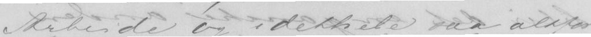Arbeide og i dethele saa altfor
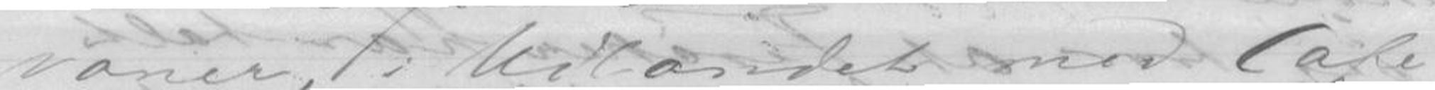vaner, i Udlandet med Café
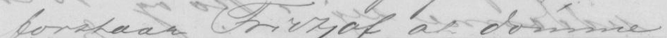forstaar Fridtjof at dømme
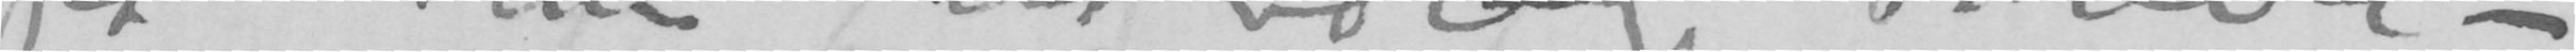på Dine elskværdige Breve –
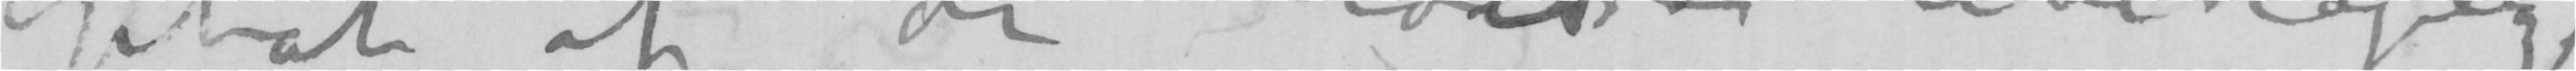optat af de høist ubehagelige
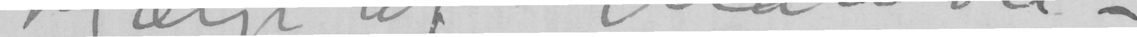Hjælp af Mamon –
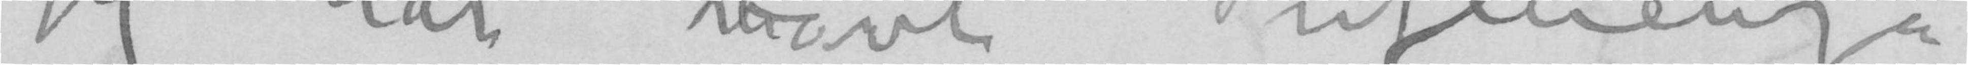Jeg har havt Influenza
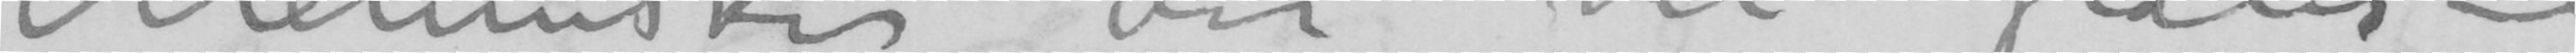Mennesker der vil fraliste
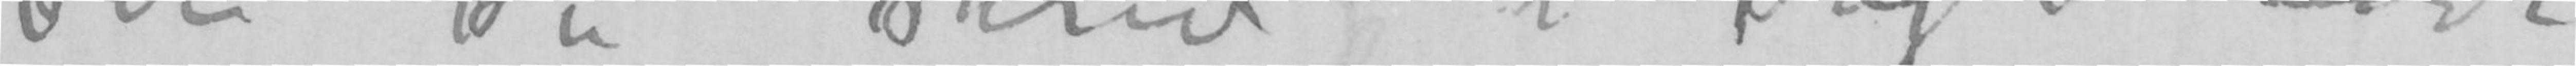Det du skrev i Dagbladet
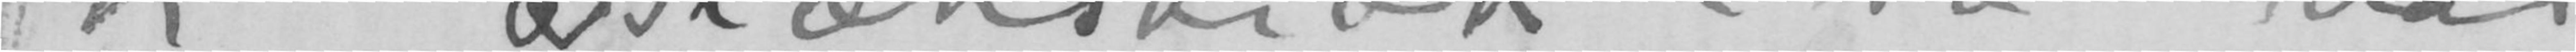for SBlækskræk – så har
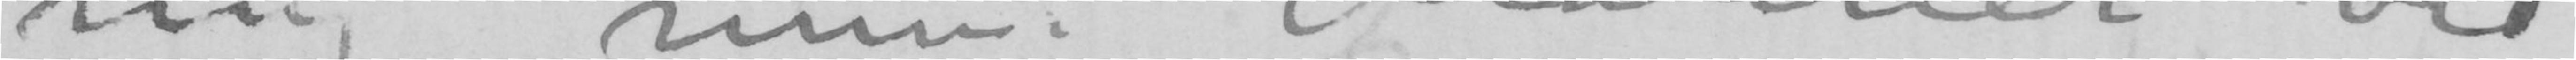mig mine Malerier ved
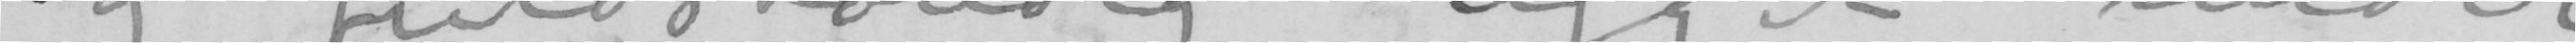og fuldstændig ligget under
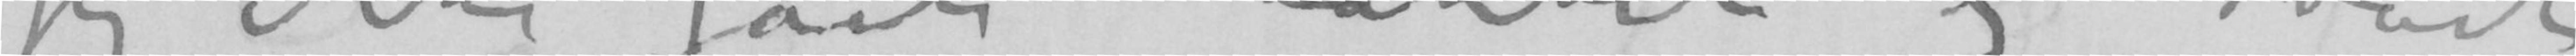jeg ikke fået takket og svart
